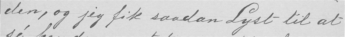den, og jeg fik saadan Lyst til at
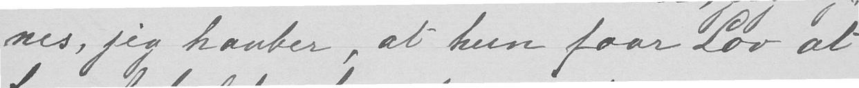nes, jeg haaber, at hun faar Lov at
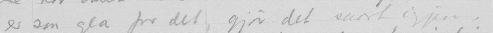er saa gla for det, gjør det snart igjen,
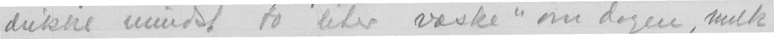drikke mindst to liter „væske” om dagen, melk
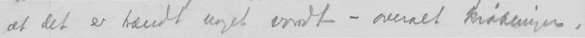at det er hændt noget vondt - overalt krøblinger,
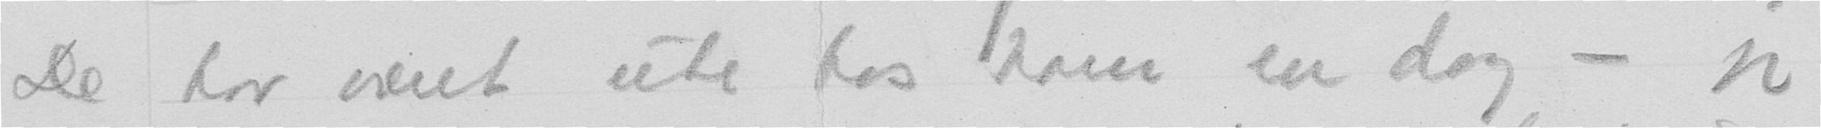De har været ute hos ham en dag - jeg
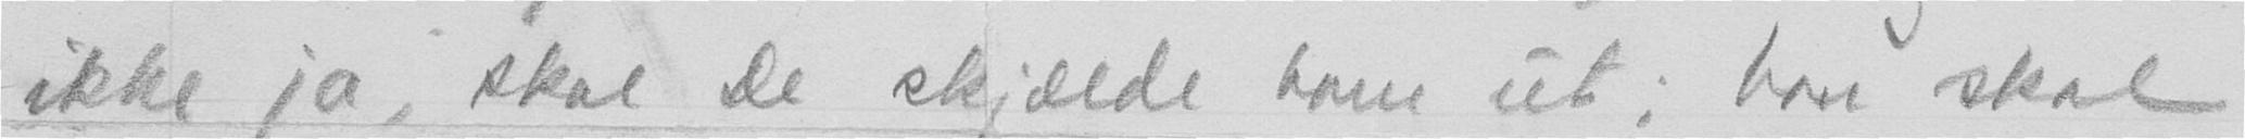ikke ja, skal de skjælde ham ut; han skal
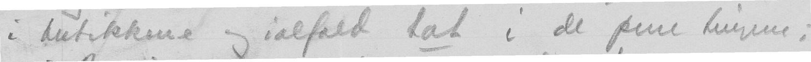i butikkene og ialfald tat i de pene tingene;
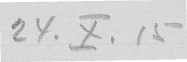24. X. 15
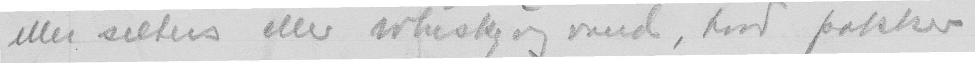eller selters eller whisky og vand, hvad pokker
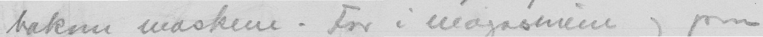bakom maskene. For i magasinene og paa
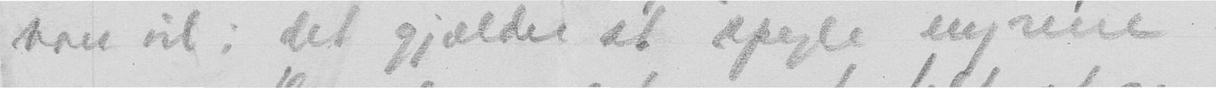han vil; det gjælder at spyle nyrene.
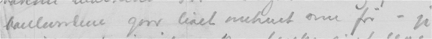boulevardene gaar livet omtrent som før - jeg
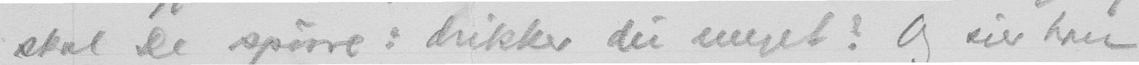skal De spørre: drikker du meget? Og sier han
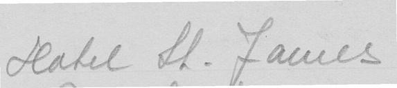Hotel St. James
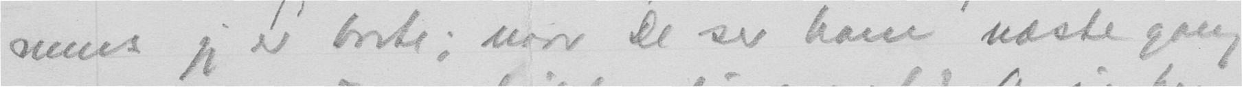mens jeg er borte; naar De ser ham næste gang
